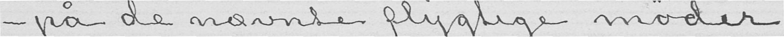- på de nævnte flygtige møder
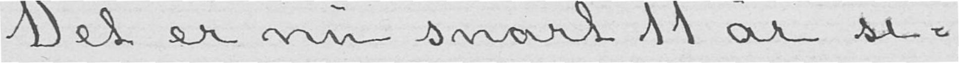Det er nu snart 11 år si-
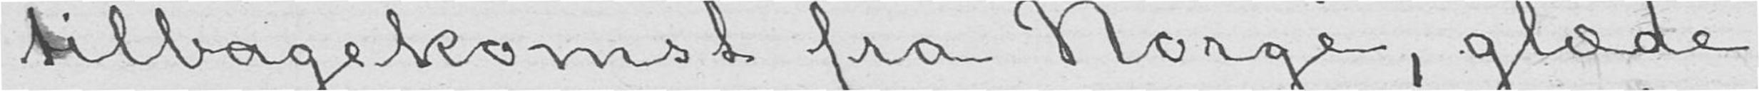tilbagekomst fra Norge, glæde
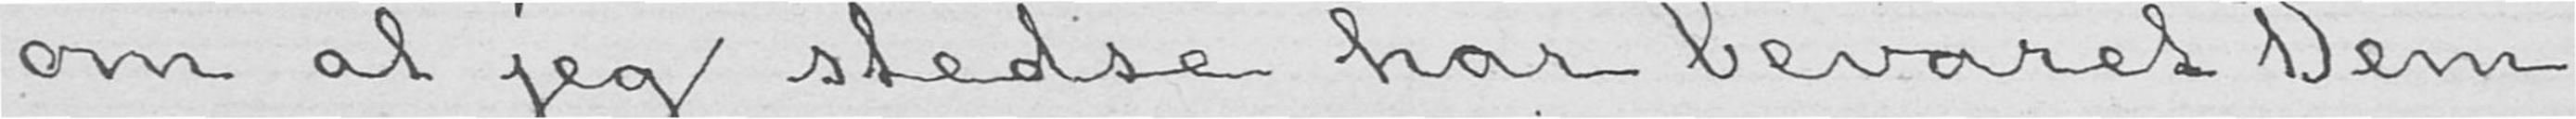om at jeg stedse har bevaret Dem
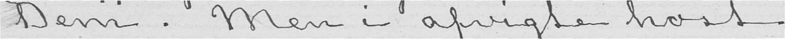Dem. Men i afvigte høst
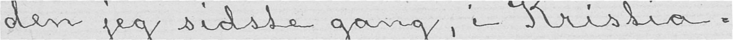den jeg sidste gang, i Kristia-
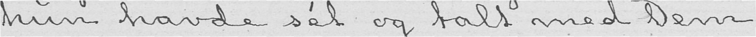hun havde sét og talt med Dem
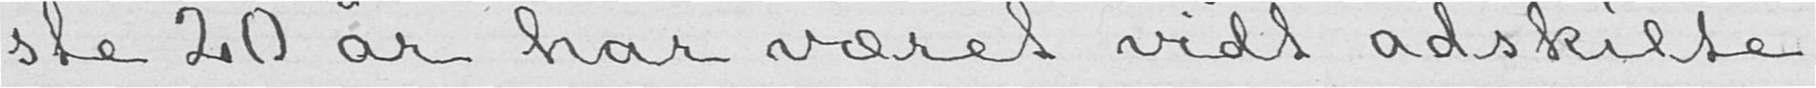ste 20 år har været vidt adskilte
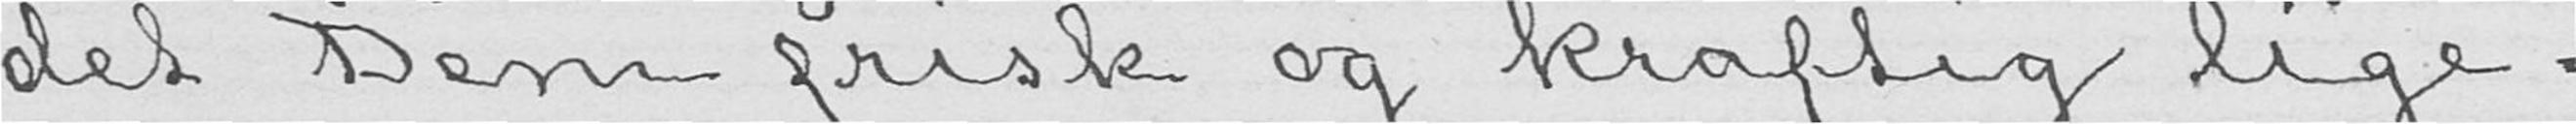det Dem frisk og kraftig lige-
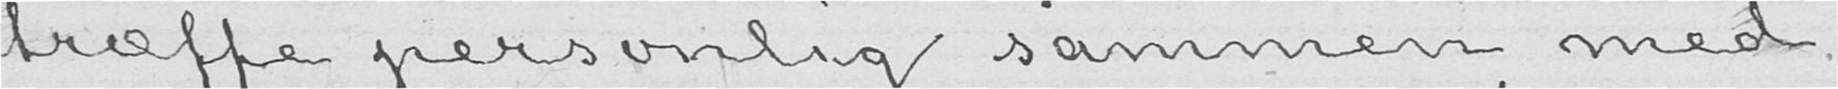træffe personlig sammen med
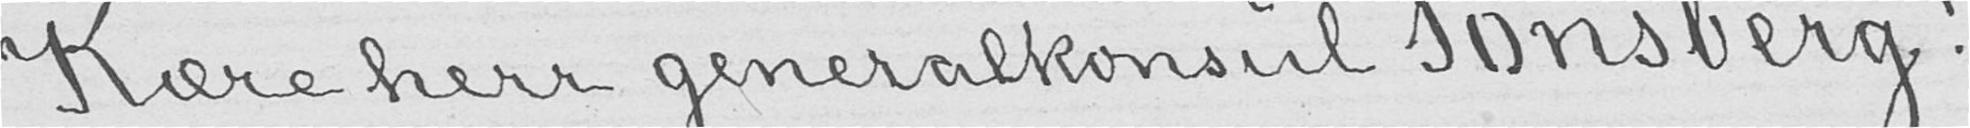Kære herr generalkonsul Tønsberg!
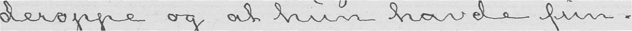deroppe og at hun havde fun-
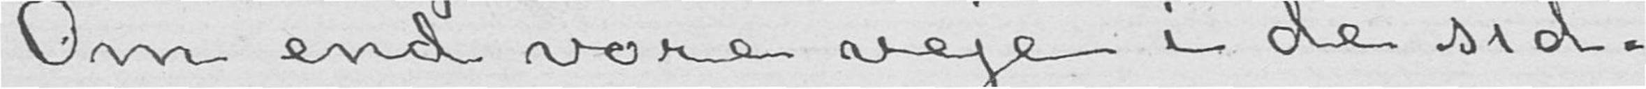Om end vore veje i de sid-
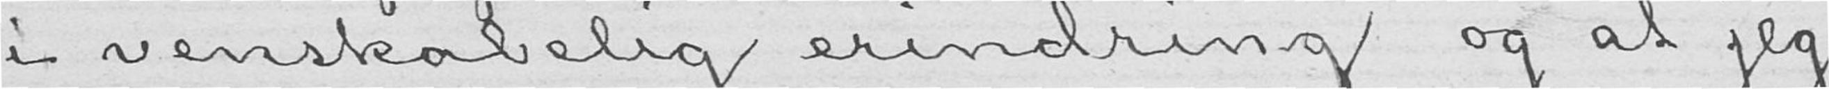i venskabelig erindring og at jeg
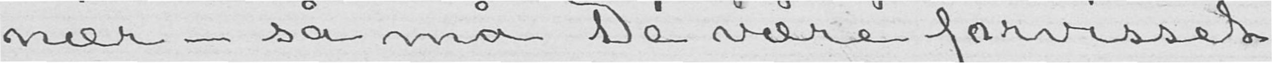nær- så må De være forvisset
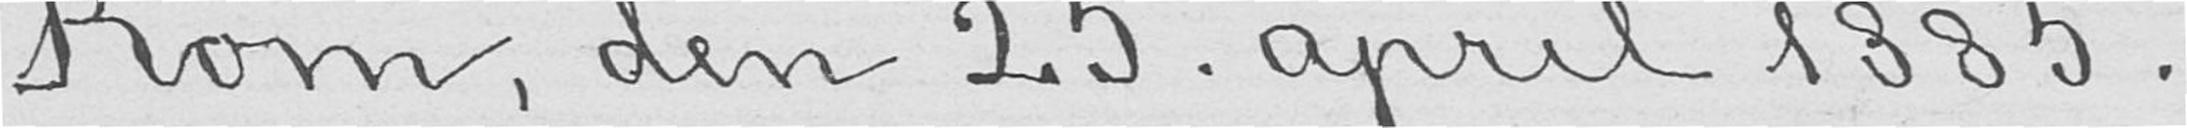Rom, den 25. april 1885.
